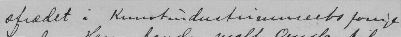strædet . Kunstindustrimuseets forrige
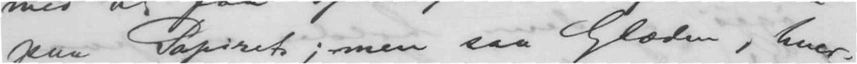paa Papiret; men saa Glæden, hver-
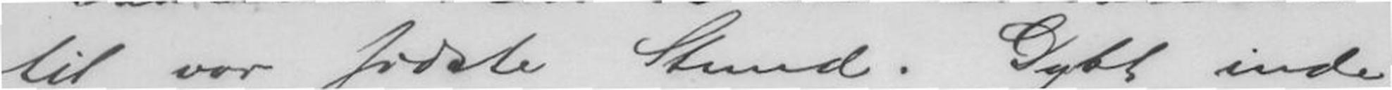til vor sidste Stund. Dybt inde
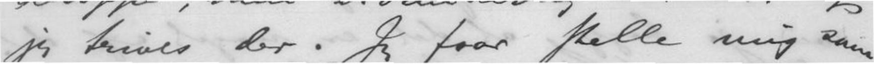jeg trives der. Jeg faar stelle mig som
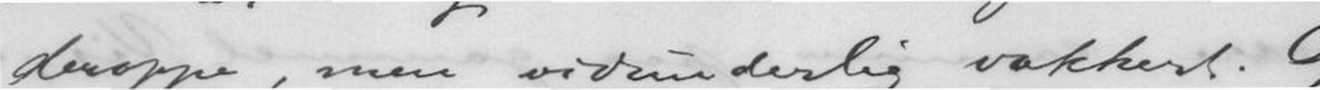deroppe, men vidunderlig vakkert. Og
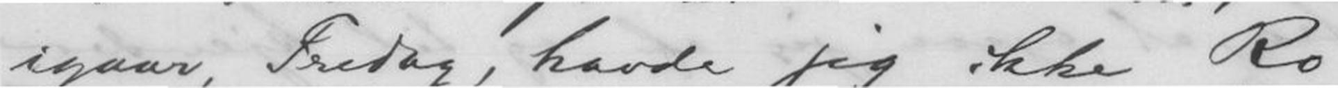igaar, Fredag, havde jeg ikke Ro
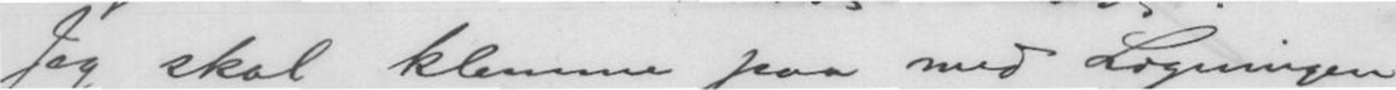Jeg skal klemme paa med Ligningen
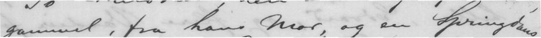gammel, fra hans Mor, og en Springdans
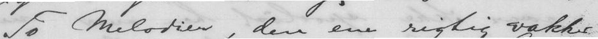To Melodier, den ene rigtig vakker,
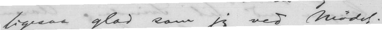ligesaa glad som jeg ved Mødet.
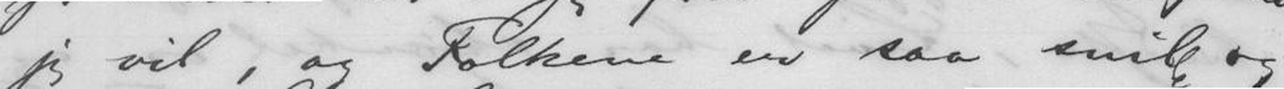jeg vil, og Folkene er saa snille og
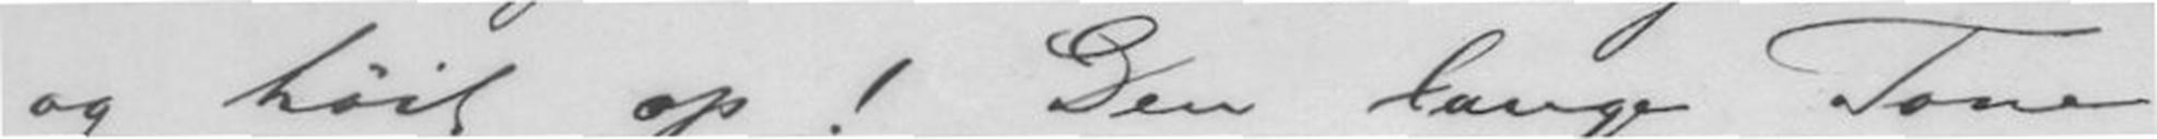og høit op! Den lange Tone
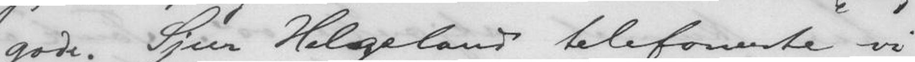gode. Sjur Helgeland telefonerte vi
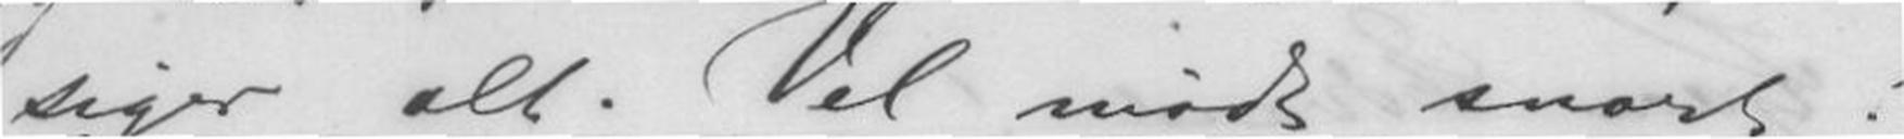siger alt. Vel mødt snart!
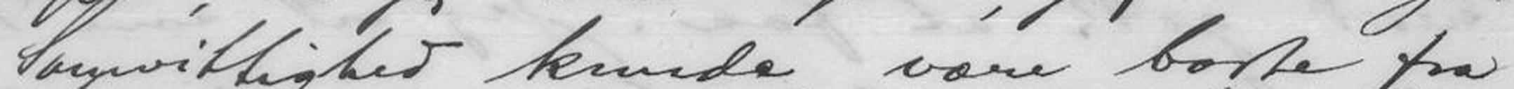Samvittighed kunde være borte fra
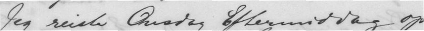Jeg reiste Onsdag Eftermiddag op
